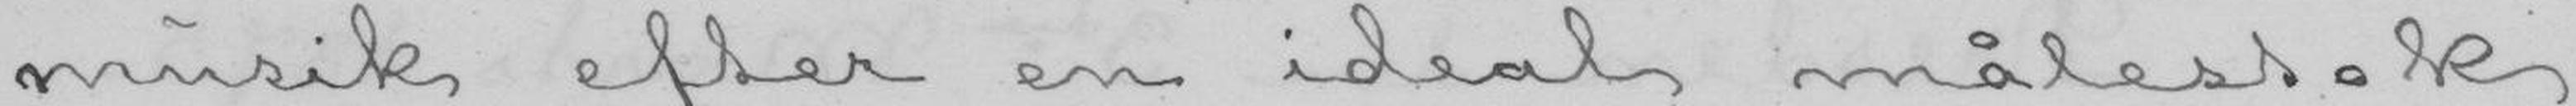musik efter en ideal målestok
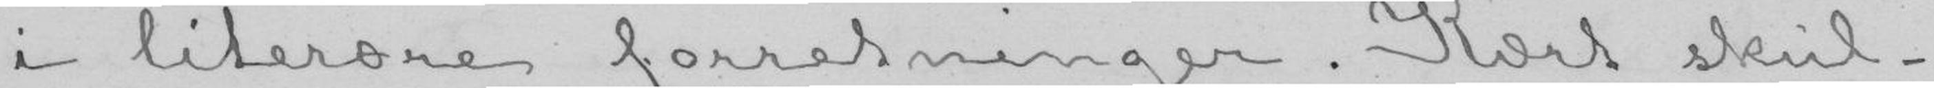i literære forretninger. Kært skul-
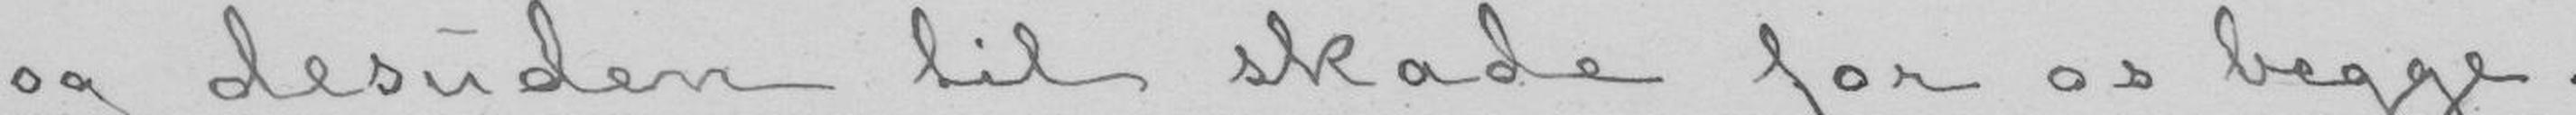og desuden til skade for os begge.
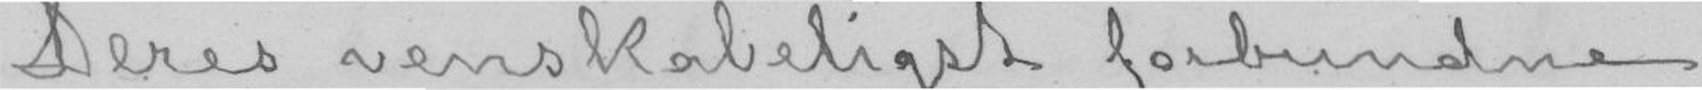Deres venskabeligst forbundne
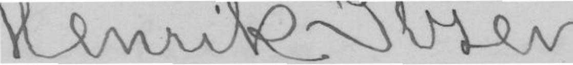Henrik Ibsen.
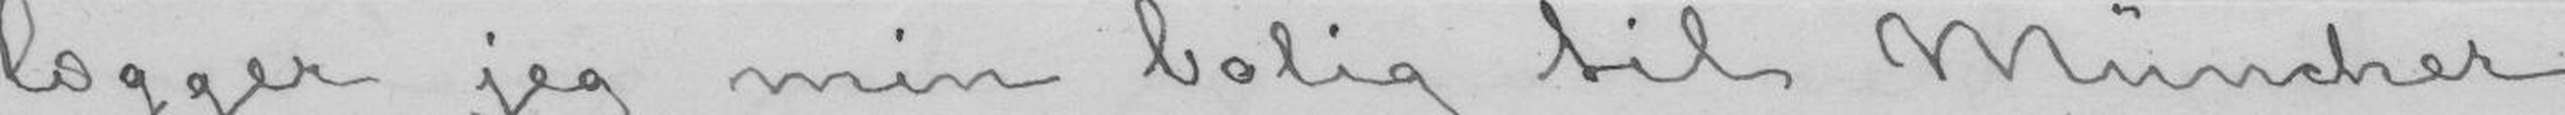lægger jeg min bolig til Müncher;
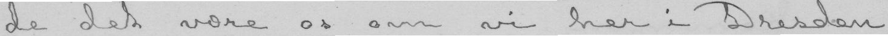de det være os om vi her i Dresden
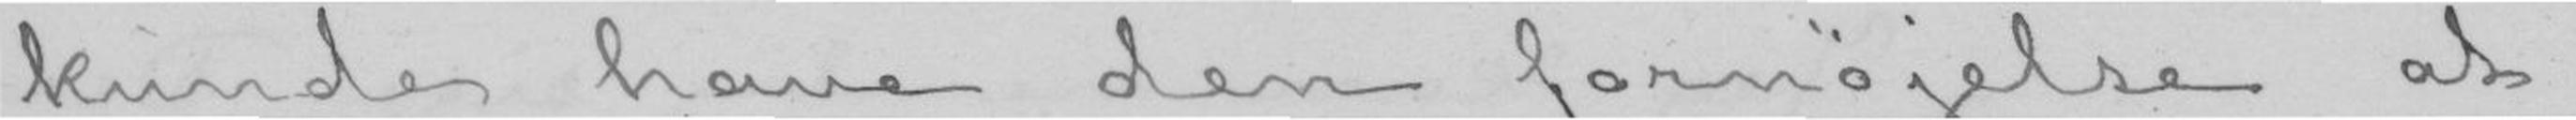kunde have den fornøjelse at
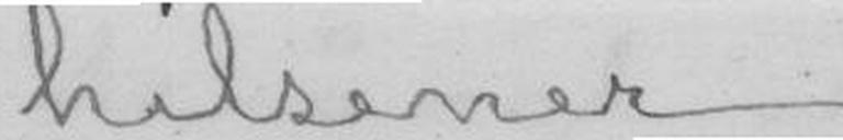hilsener.
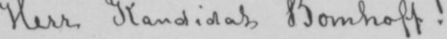Herr Kandidat Bomhoff!
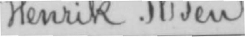Henrik Ibsen.
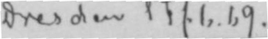Dresden 17/6. 69.
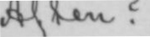Aften?
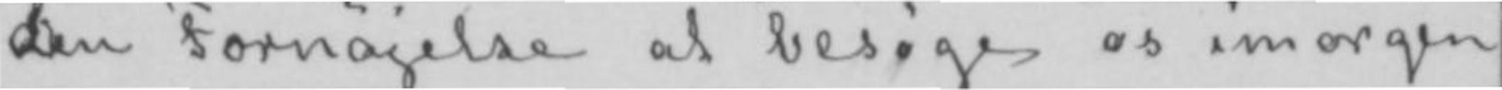den Fornøjelse at besøge os imorgen
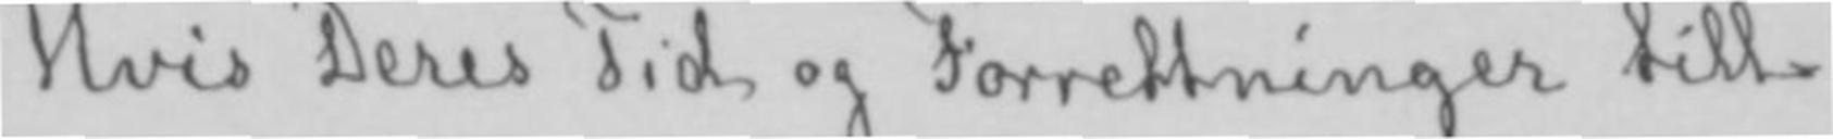Hvis Deres Tid og Forrettninger till-
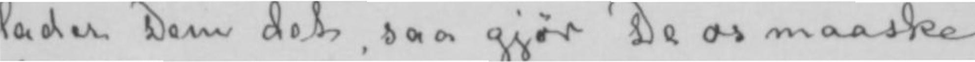lader Dem det, saa gjør De os maaske
